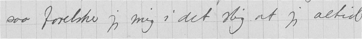saa forelsker jeg mig i det slig at jeg altid
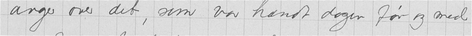anger over det, som var hændt dagen før og med
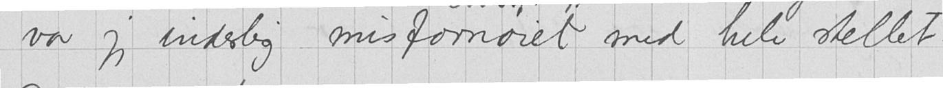var jeg inderlig misfornøiet med hele stellet.
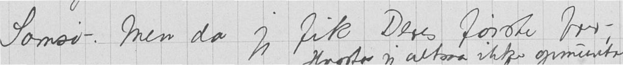Samsø. Men da jeg fik Deres første brev -,
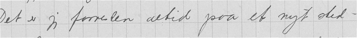Det er jeg forresten altid paa et nyt sted -
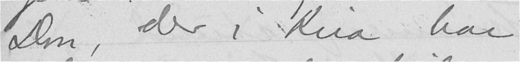Don, der i Kria har
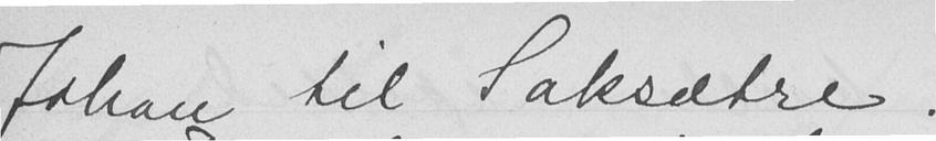Johan til Saksætre.
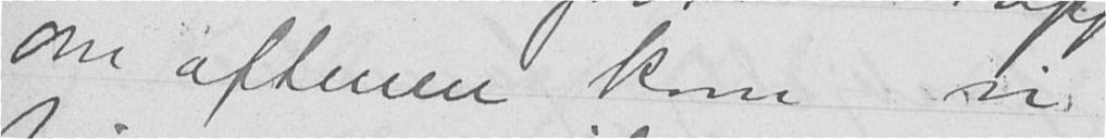Om aftenen kom vi
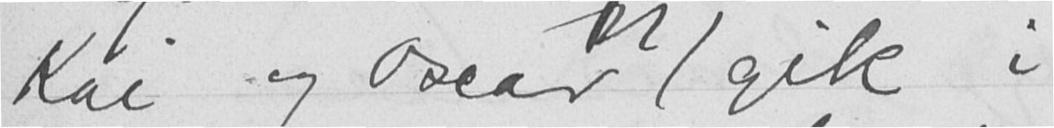Kai og Oscar gik i
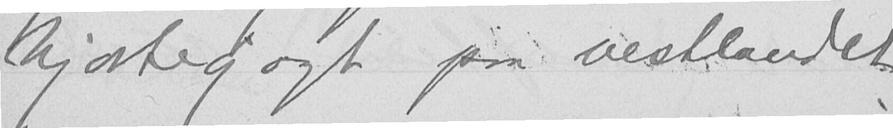hjortejagt paa vestlandet
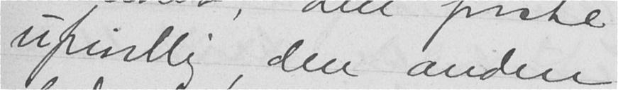ufrivillig, den anden
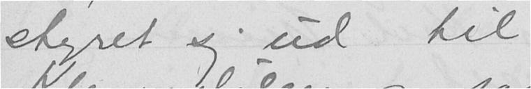styret sig ud til
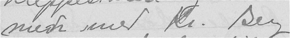men med Kr. Berg
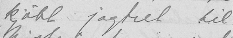kjøbt jagtret til
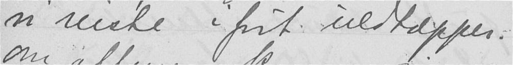vi reiste iført uldtæpper.
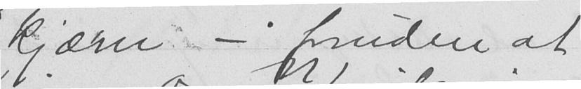kjærn - foruden at
